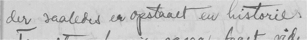der saaledes er opstaaet en historie.
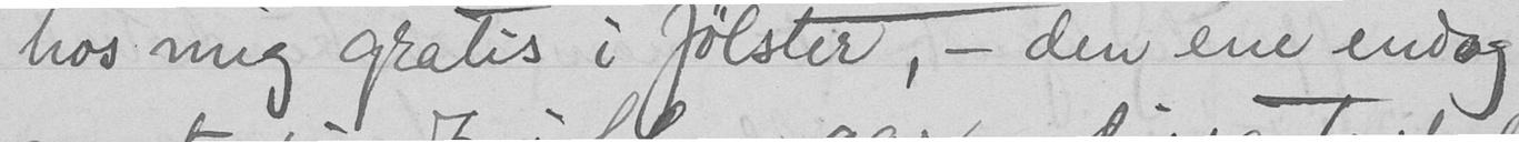hos mig gratis i Jølster, - den ene endog
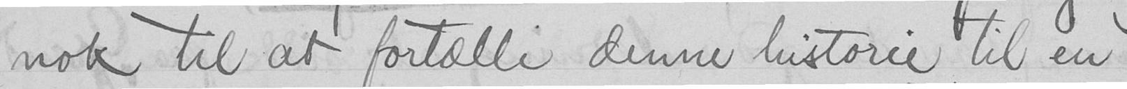nok til at fortælle denne historie til en
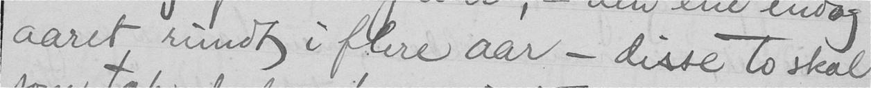aaret rundt i flere aar - disse to skal
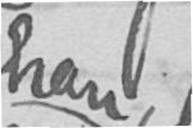han
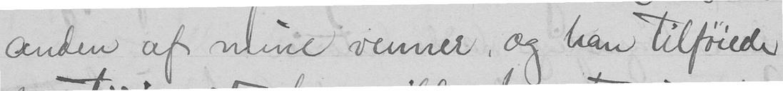anden af nline venner, og han tilføiede
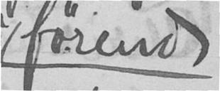førend
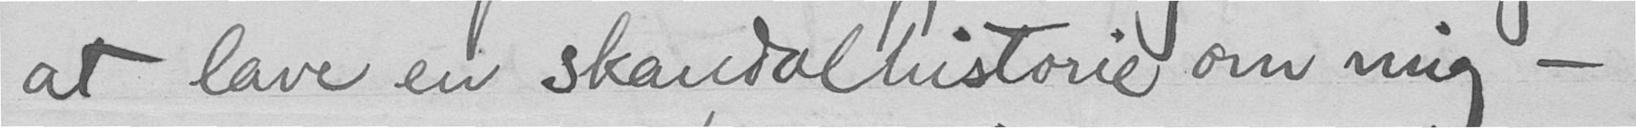at lave en skandalhistorie om mig -
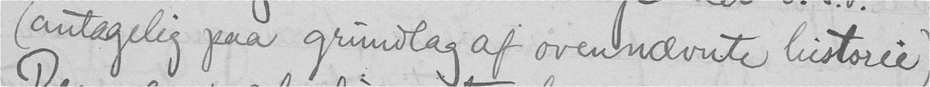(antagelig paa grundlag af ovennævnte historie)
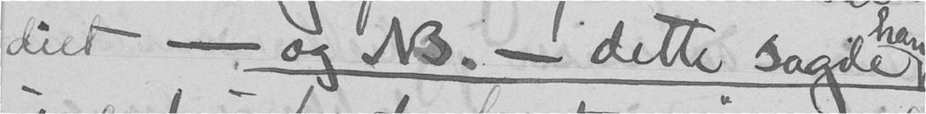diet - og NB. - dette sagde
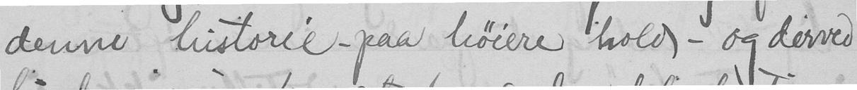denne historie - paa høiere hold, - og derved
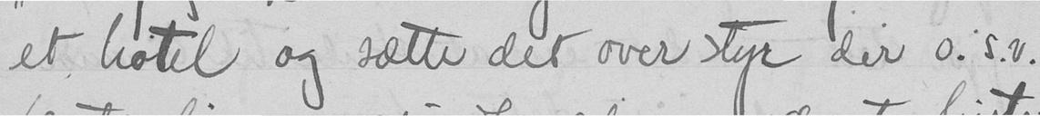et hotel og sætte det over styr der o.s.v.
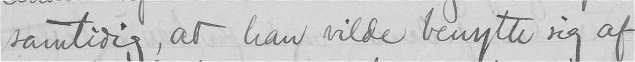samtidig, at han vilde benytte sig af
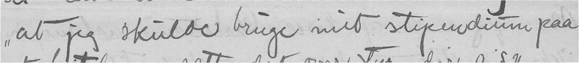"at jeg skulde bruge mit stipendium paa
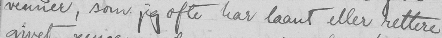venner, som jeg ofte har laant eller rettere
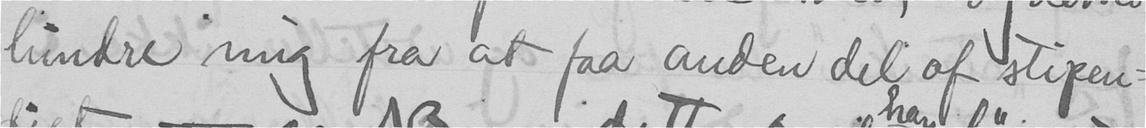hundre mig fra at faa anden del af stipen-
